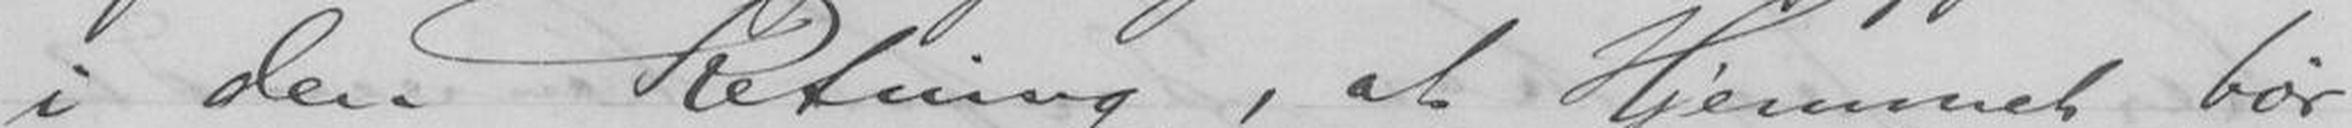i den Retning, at Hjemmet bør
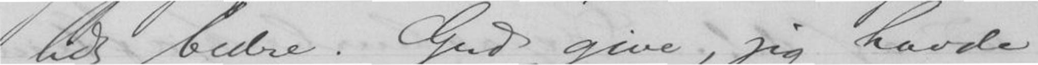lidt bedre. Gud give, jeg havde
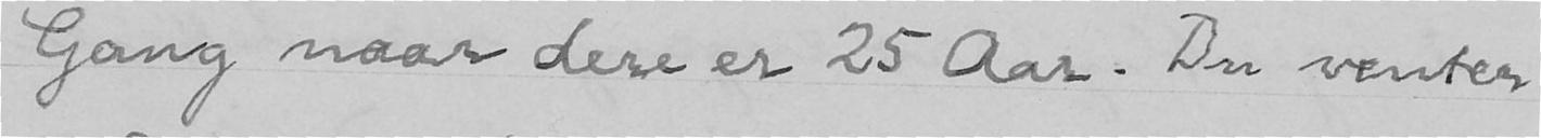Gang naar dere er 25 Aar. Du venter
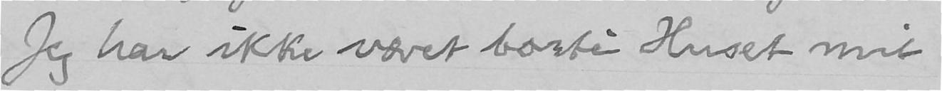Jeg har ikke været borti Huset mit
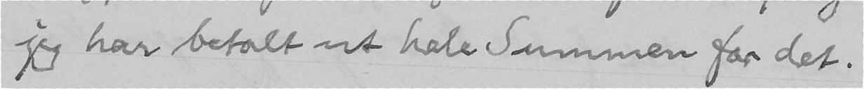jeg har betalt ut hele Summen for det.
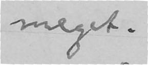meget.
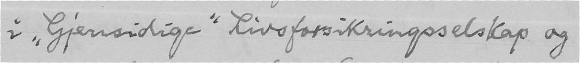i "Gjensidige" Livsforsikringsselskap og
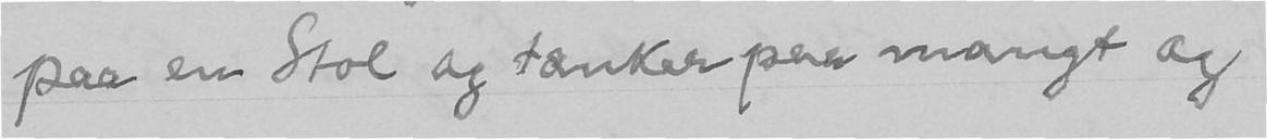paa en Stol og tænker paa mangt og
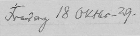Fredag 18 Oktbr. 29.
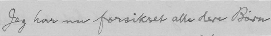Jeg har nu forsikret alle dere Børn
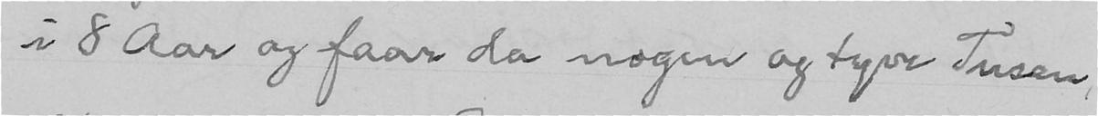i 8 Aar og faar da nogen og tyve Tusen,
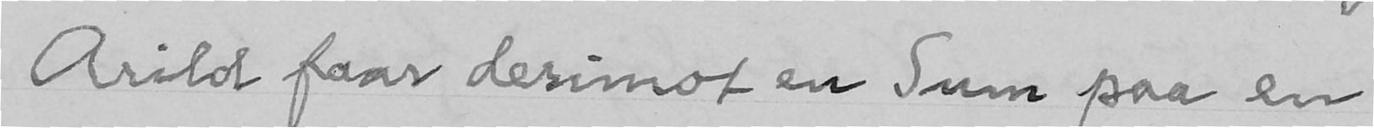Arild faar derimot en Sum paa en
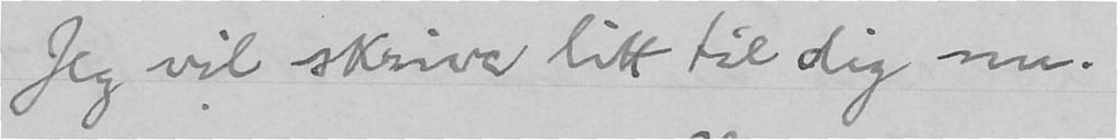Jeg vil skrive litt til dig nu.
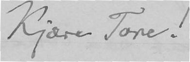Kjære Tore!
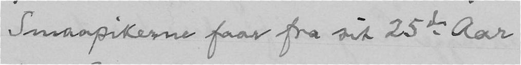Smaapikerne faar fra sit 25te Aar
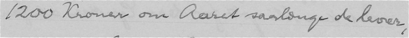1200 Kroner om Aaret saalænge de lever,
